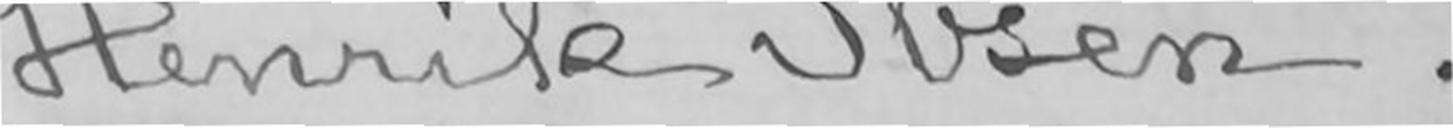Henrik Ibsen.
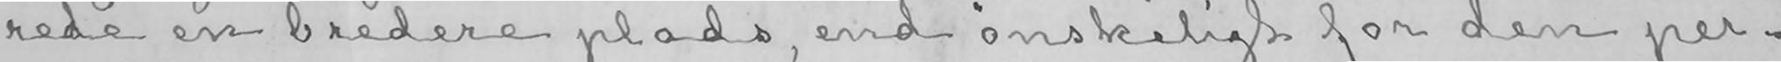rede en bredere plads, end ønskeligt for den per-
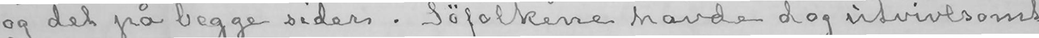og det på begge sider. Søfolkene havde dog utvivlsomt
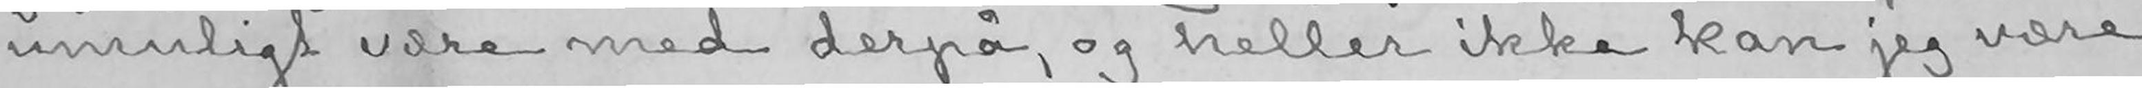umuligt være med derpå, og heller ikke kan jeg være
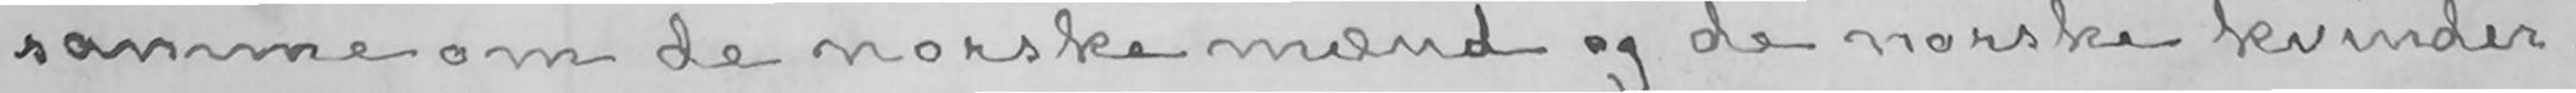samme om de norske mænd og de norske kvinder.
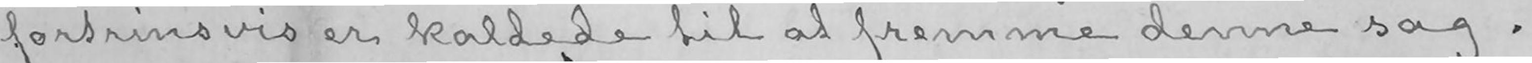fortrinsvis er kaldede til at fremme denne sag.
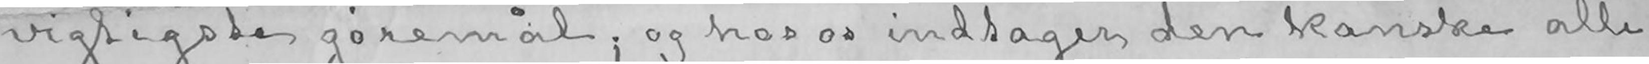vigtigste gøremål; og hos os indtager den kanske alle-
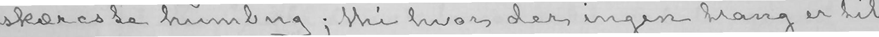skæreste humbug; thi hvor der ingen trang er til
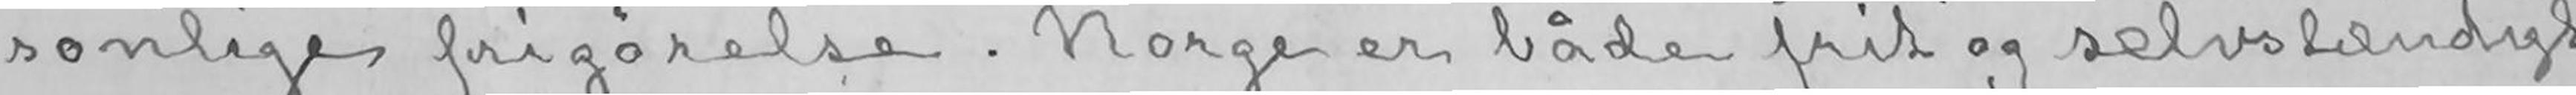sonlige frigørelse. Norge er både frit og selvstændigt
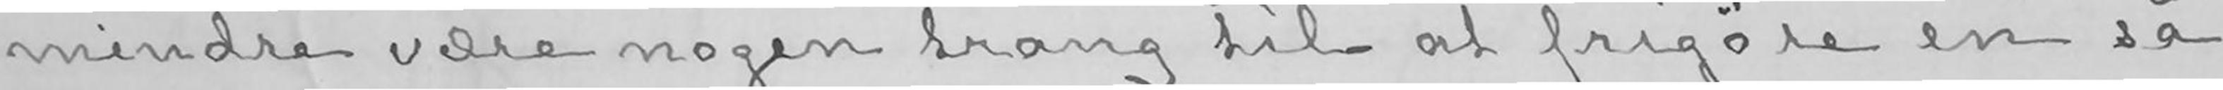mindre være nogen trang til at frigøre en så
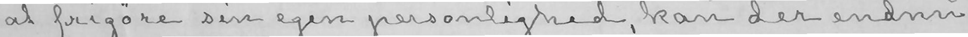at frigøre sin egen personlighed, kan der endnu
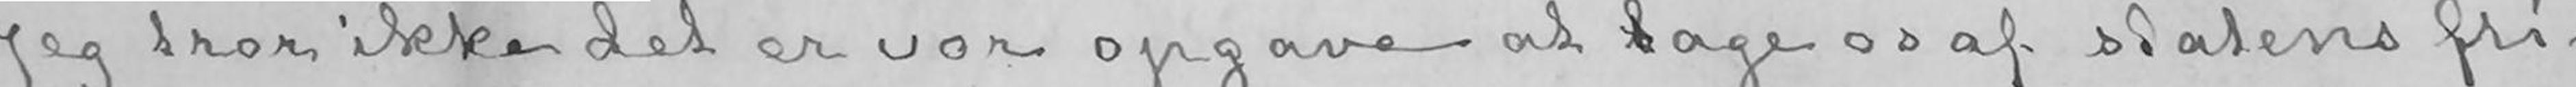Jeg tror ikke det er vor opgave at tage os af statens fri-
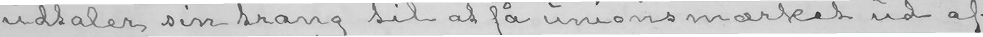udtaler sin trang til at få unionsmærket ud af
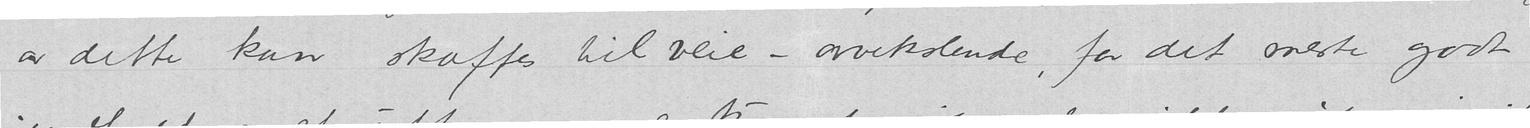av dette kan skaffes til veie – avvekslende, for det meste godt
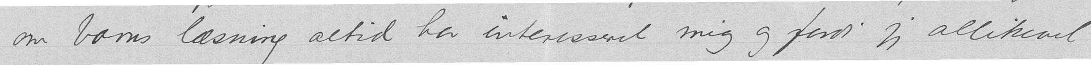om barns læsning altid har interesseret mig og fordi jeg allikevel
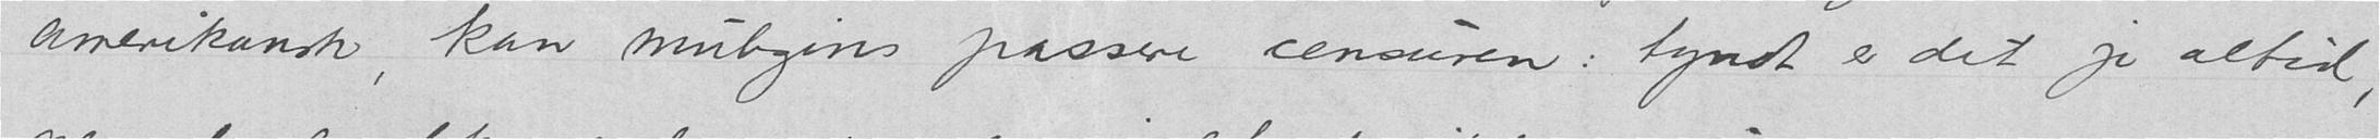amerikansk, kan muligens passere censuren: tyndt er det jo altid,
